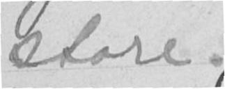store.
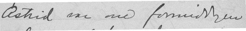Astrid var om formiddagen
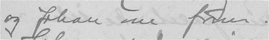og Johan om form.
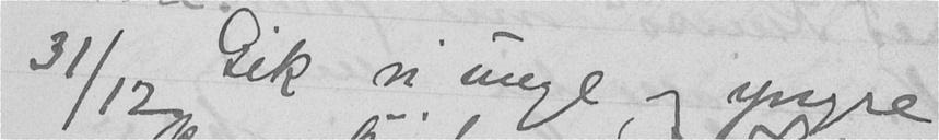31/12 Gik vi unge og yngre
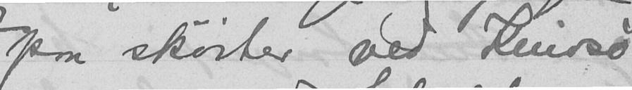paa skøiter ved Knivsø
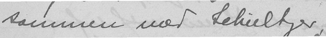sammen med Lehieltyer
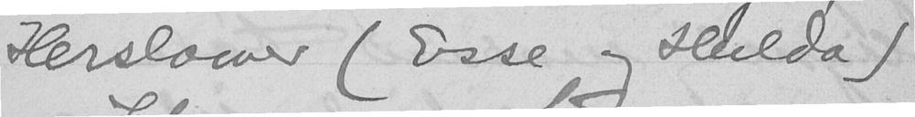Herslower (Esse og Hulda)
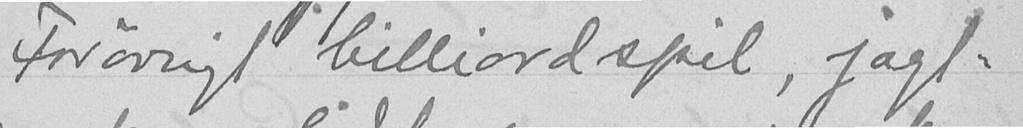Forøvrigt billiardspil, jagt-
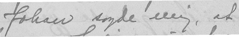Johan sagde mig, at
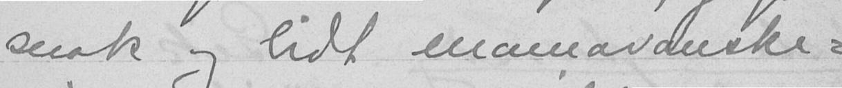snak og lidt mamavanske-
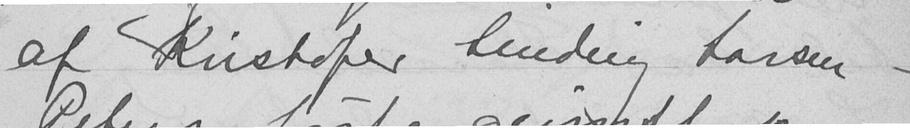af Kristofer Sinding Larsen -
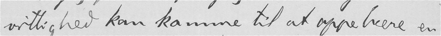vittighed kan komme til at oppebære en
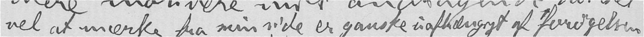vel at mærke fra min side er ganske uafhængigt af forøgelsen
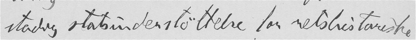stadig statsunderstøttelse for retshistoriske
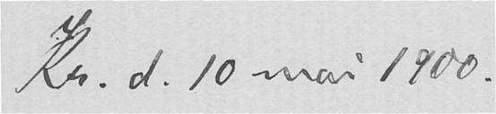Kr. d. 10 mai 1900.
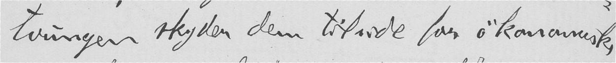tvungen skyder dem tilside for økonomiske.
